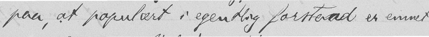paa, at populært i egentlig forstand er emnet
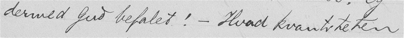dermed Gud befalet! – Hvad kvantiteten
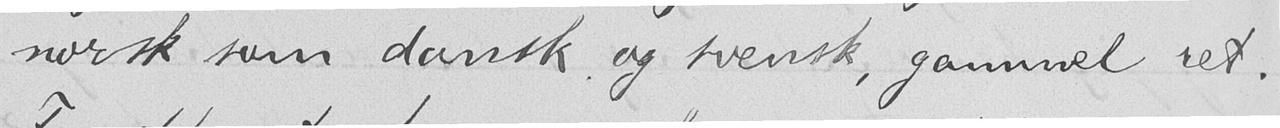norsk som dansk og svensk, gammel ret.
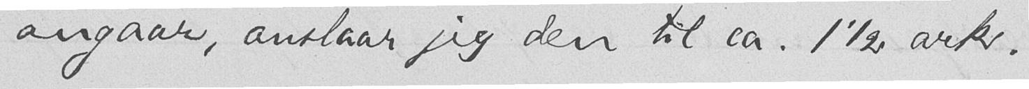angaar, anslaar jeg den til ca. 1 1/2 ark.
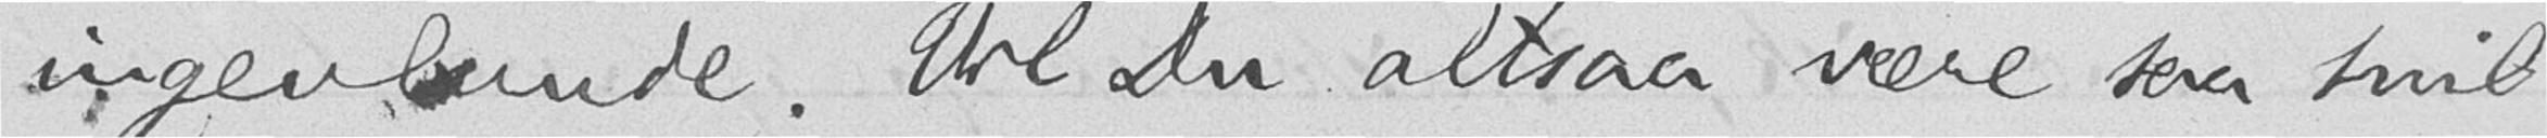ingenlunde. Vil Du altsaa være saa snil
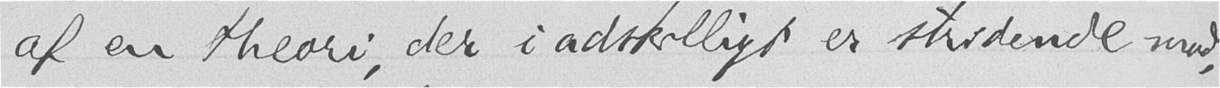af en theori, der i adskilligt er stridende mod,
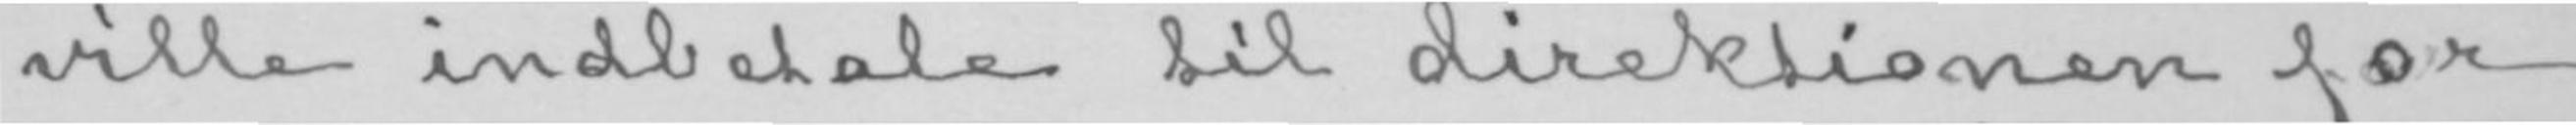ville indbetale til direktionen for
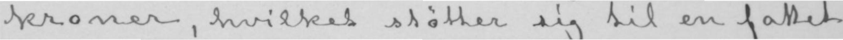kroner, hvilket støtter sig til en fattet
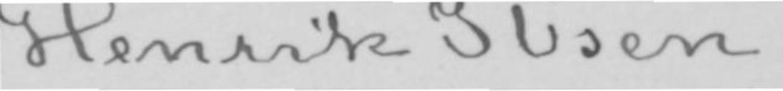Henrik Ibsen.
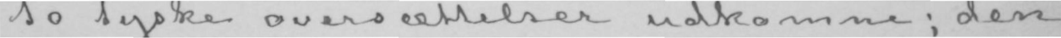to tyske oversættelser udkomne; den
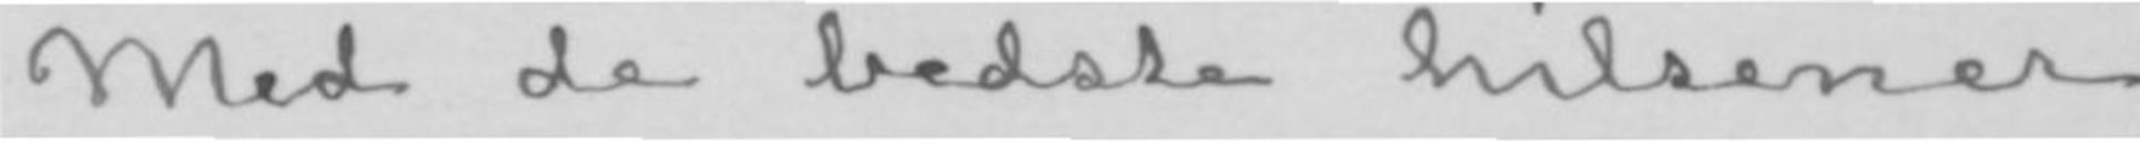Med de bedste hilsener
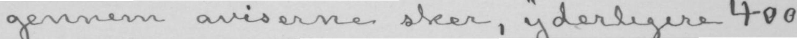gennem aviserne sker, yderligere 400
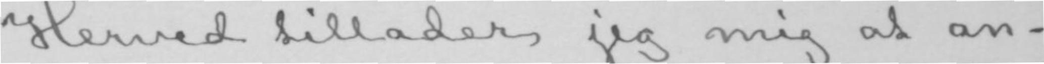Herved tillader jeg mig at an-
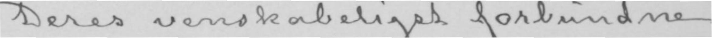Deres venskabeligst forbundne
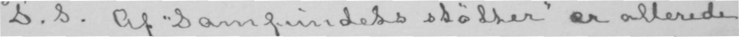P. s. Af «Samfundets støtter» er allerede
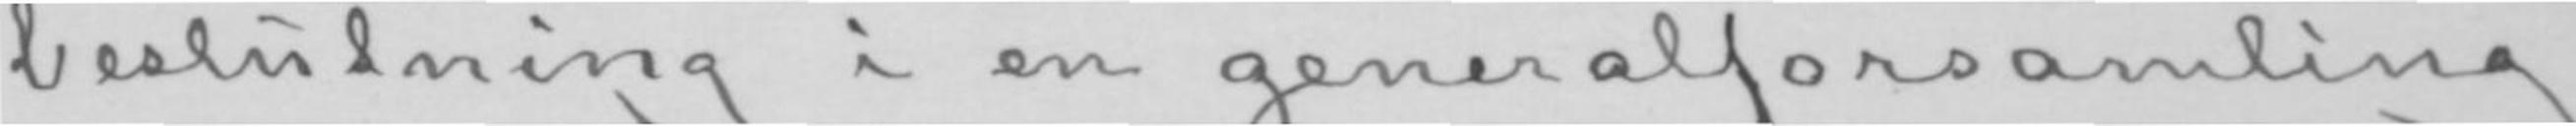beslutning i en generalforsamling.
